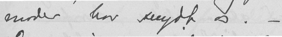moder har snydt os. -
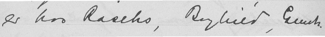er hos Raschs, Borghild, Gunh.
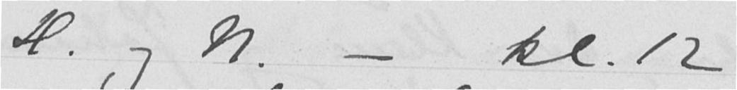H. og N. - kl. 12
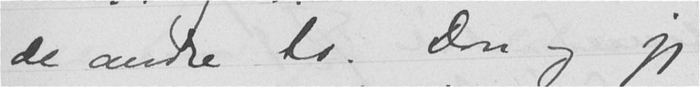de andre to. Don og jeg
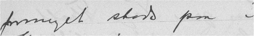formeget stads paa i
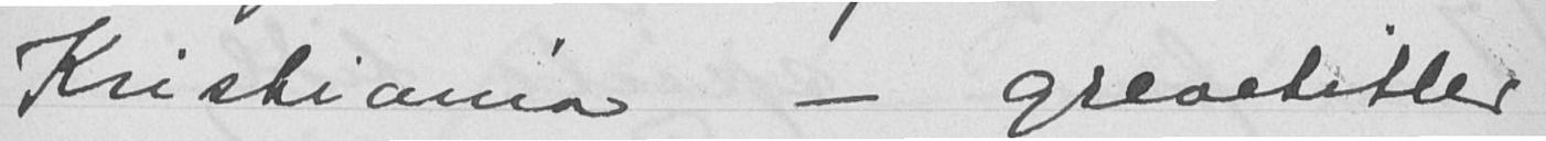Kristiania - grevetitler
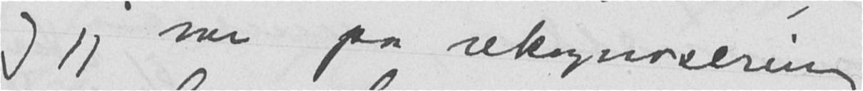og jeg var paa rekognosering
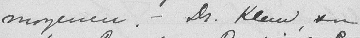morgenen - Dr. Klem, som
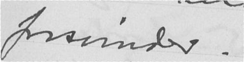forsvinder.
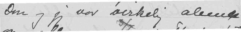Don og jeg var virkelig alene
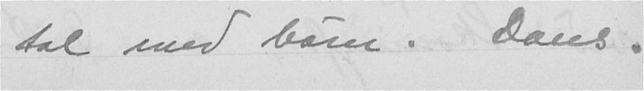tal med børn. Dans.
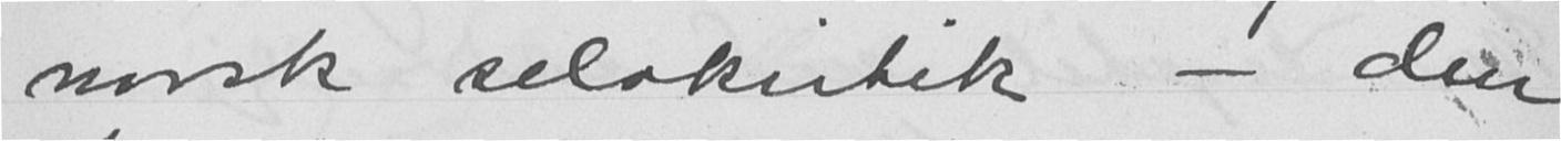norsk selvkritik - den
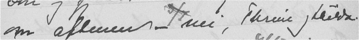om aftenen - nei, Thrine og Hulda.
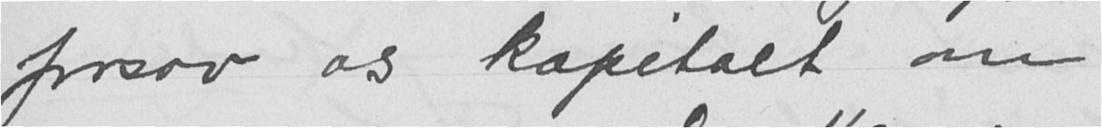forsov os kapitalt om
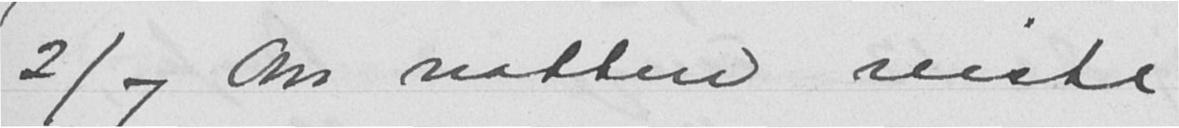2/7 Om natten reiste
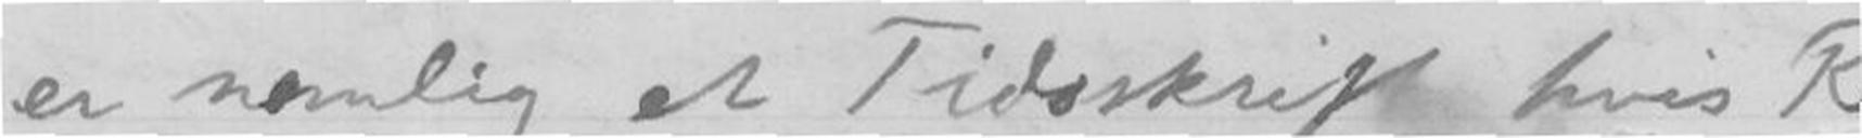er næmlig et Tidskrift hvis Red.
